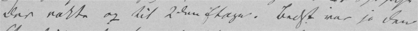der rakte op til 2den Etage. Bedst var jo den
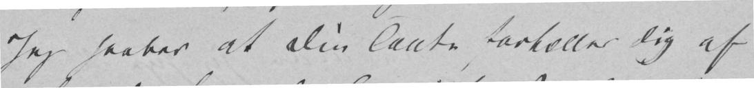Jeg haaber at Din Tante fortæller Dig af
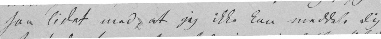saa lidet med, at jeg ikke kan meddele Dig
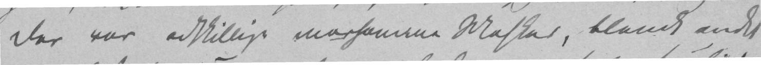Der var adskillige morsomme Masker, blandt andet
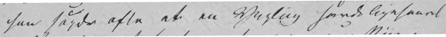han sagde ofte at en Yngling havde ligesaavel
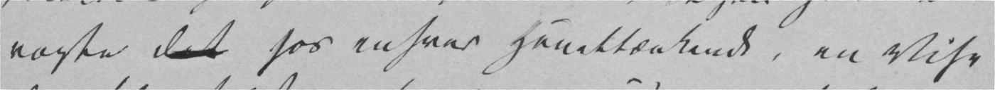vagte det hos enhver Honettænkende, en Vise
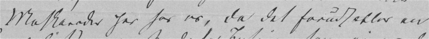Maskerader her hos os, da det forudsætter en
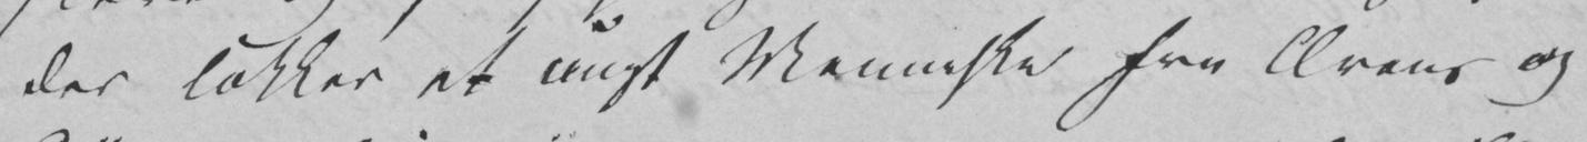der lokker et ungt Menneske fra Ærens og
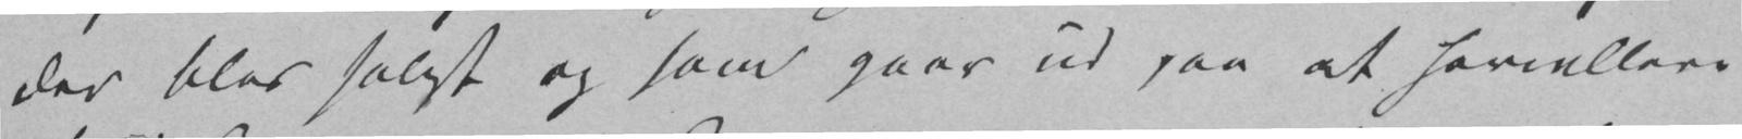der blev solgt og som gaar ud paa at harcellere
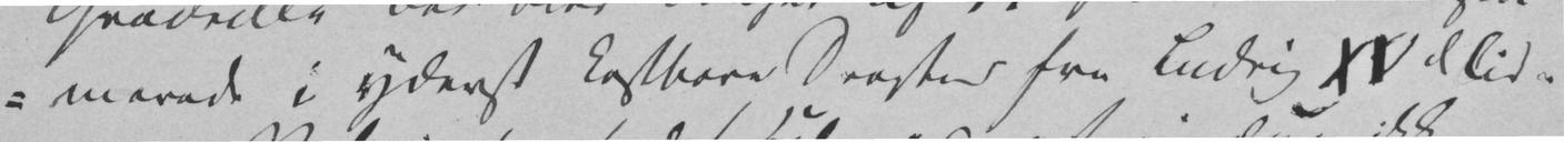merede i yderst kostbare Dragter fra Ludvig XVde Tid.
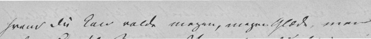hvem Du kan volde megen, megen Glæde, men
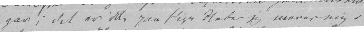gav; det er ikke paa slige Steder jeg morer mig –
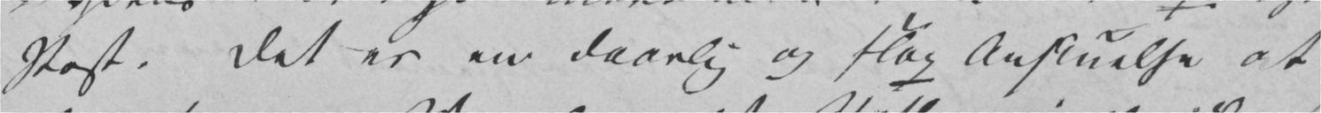Post. Det er en daarlig og slap Anskuelse at
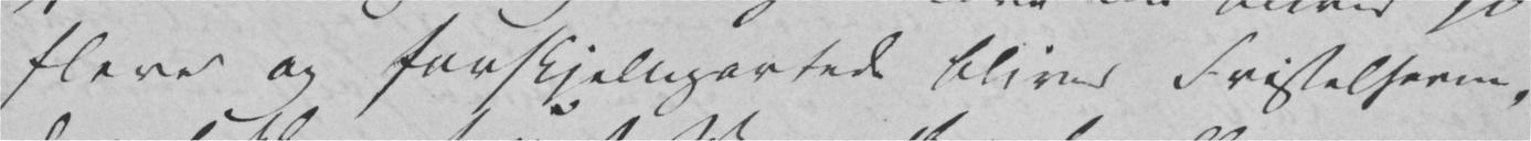flere og forskjelligartede bliver Fristelserne,
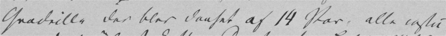Qvadrille der blev danset af 14 Par, alle costu-
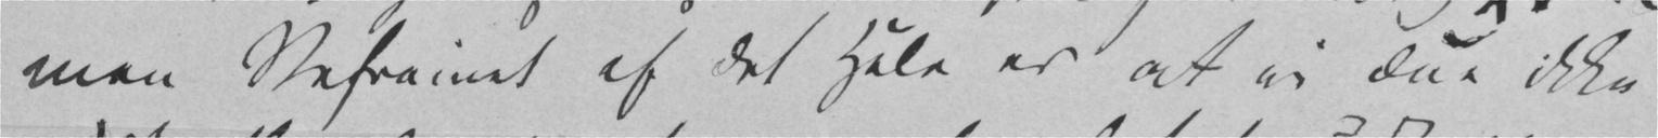men Refrainet af det Hele er at vi due ikke
